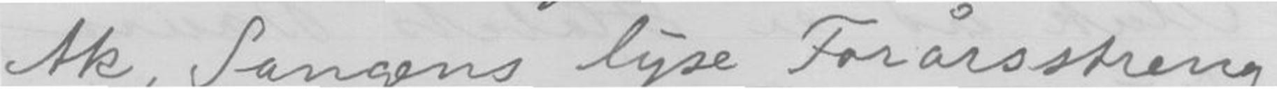Ak, Sangens lyse Forårsstreng
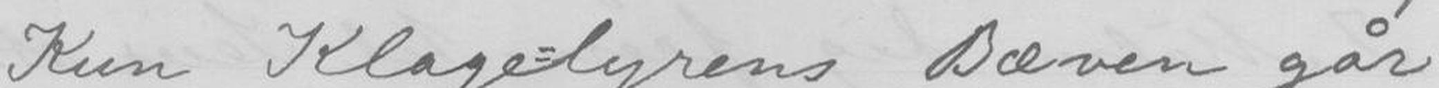Kun Klage=lyrens Bæven går
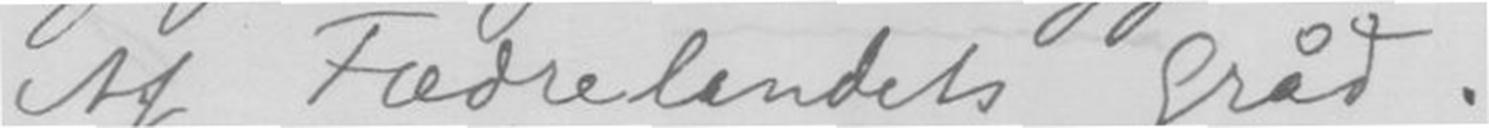Af Fædrelandets Gråd.
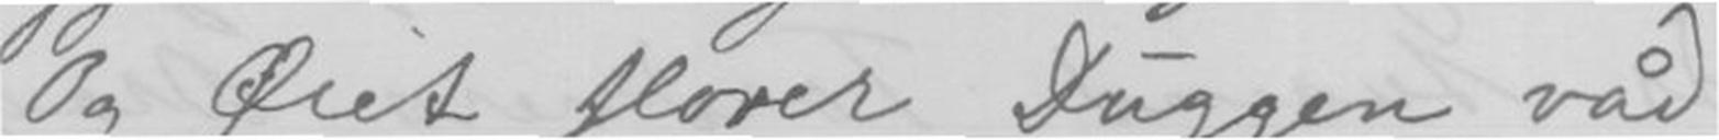Og Øiet florer Duggen våd
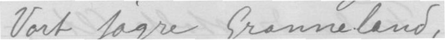Vort fagre Granneland,
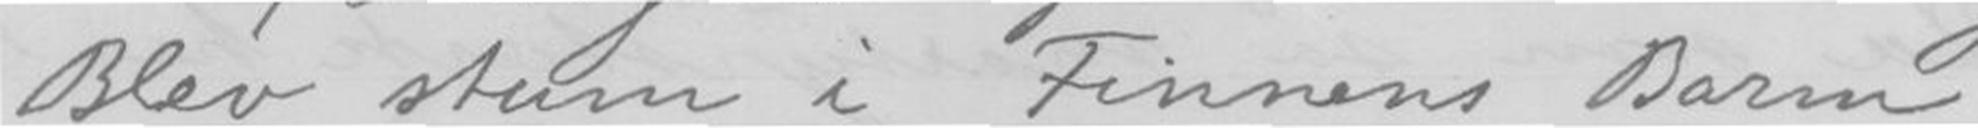Blev stum i Finnens Barm,
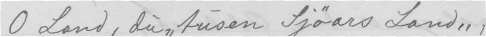O Land, du "tusen Sjøars Land",
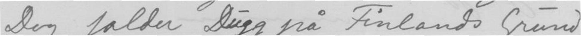Dog falder Dugg på Finlands Grund
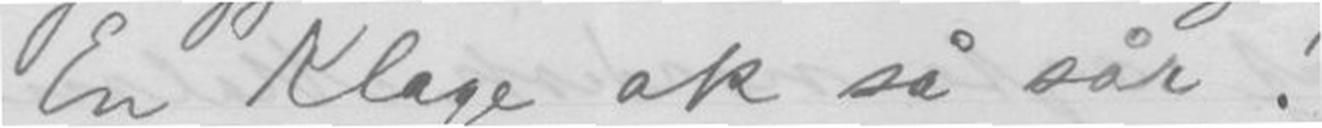En Klage ak så sår!
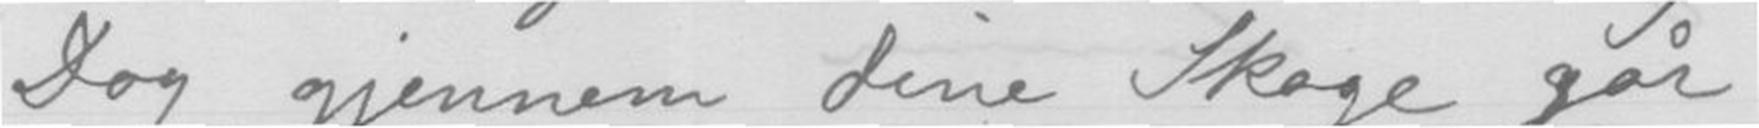Dog gjennem Dine Skoge går
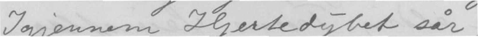Igjennem Hjertedybet sår,
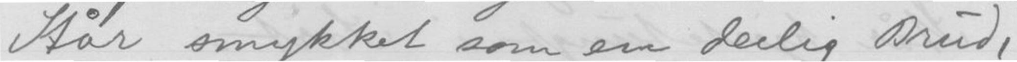Står smykket som en deilig Brud,
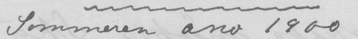Sommeren ano 1900
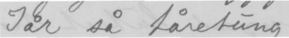Iår så tåretung.
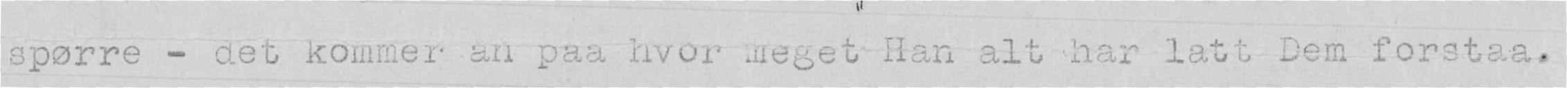spørre - det kommer an paa hvor meget Han alt har latt Dem forstaa.
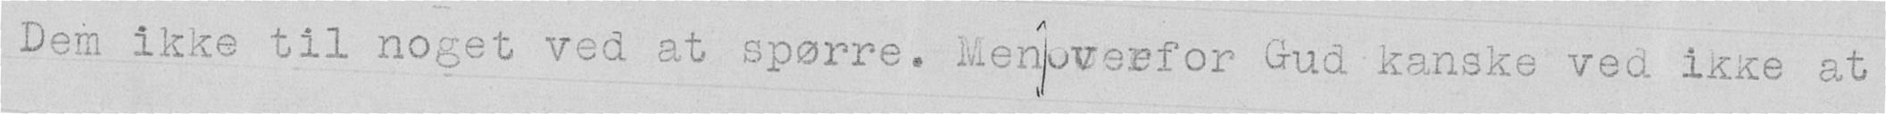Dem ikke til noget ved at spørre. Menoverfor Gud kanske ved ikke at
